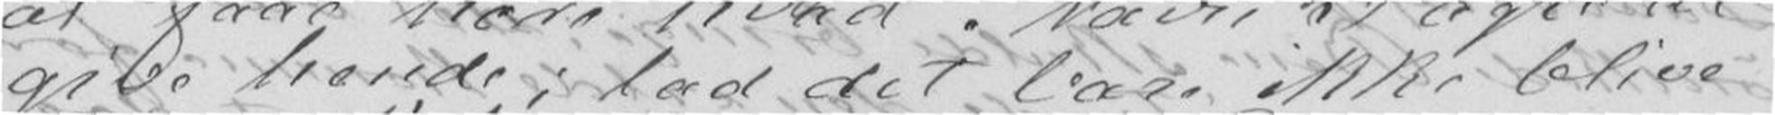give hende; lad det bare ikke blive
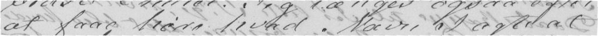at faae høre hvad Navn I agte at
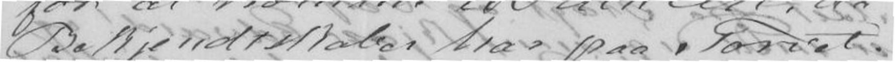Bekjendtskaber har paa Torvet.
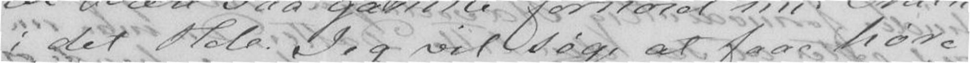i det Hele. Jeg vil søge at faae høre
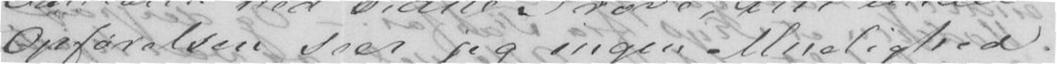Opførelsen seer jeg ingen Mulighed,
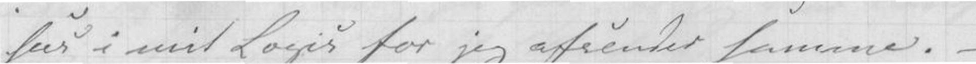sees i mit Logis for jeg afsender samme. -
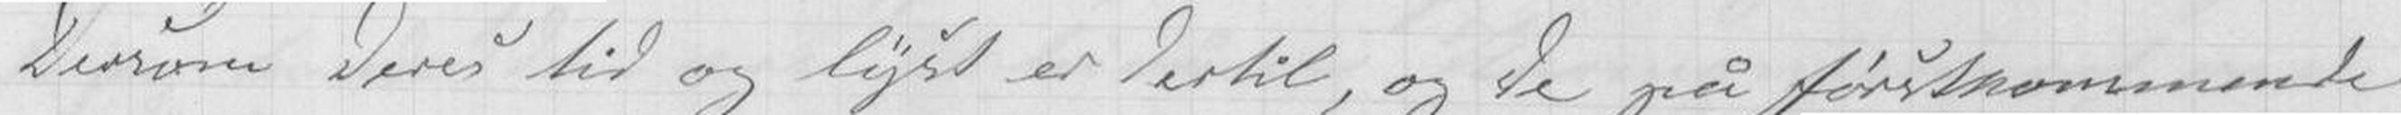Dersom Deres tid og lyst er Dertil, og De på førstkommende
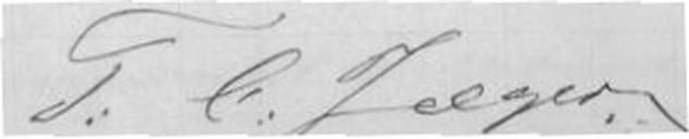T: C: Jæger
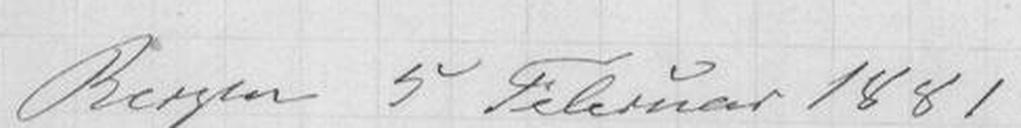Bergen 5 Februar 1881
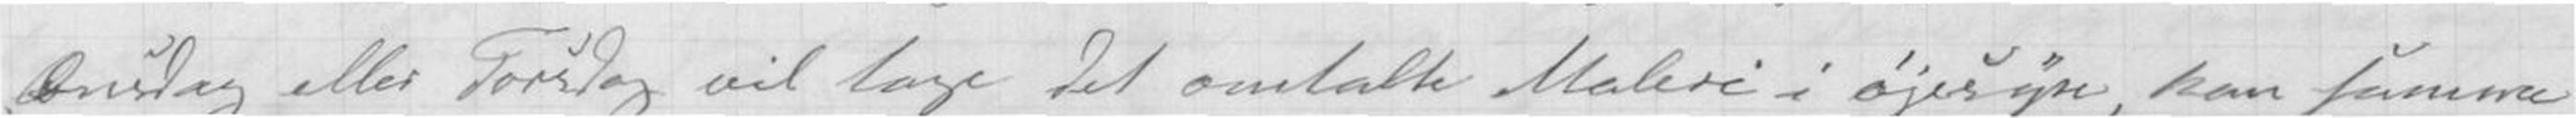Onsdag eller Torsdag vil lage Det omtalte Maleri i øjesyn, kan samme
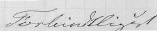Forbindligst
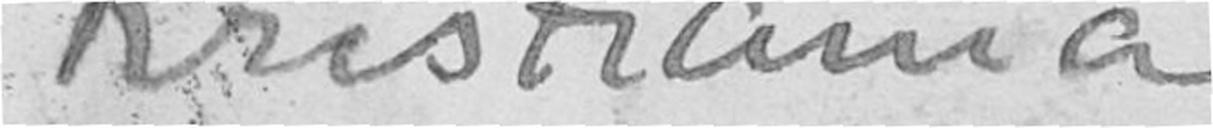Kristiania
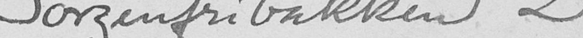Sorgenfribakken 2
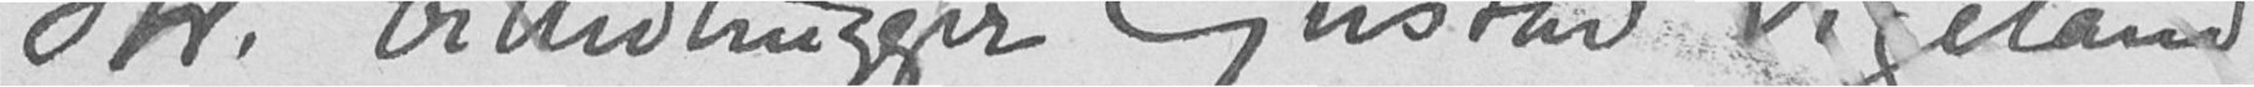Hr. billedhugger Gustav Vigeland
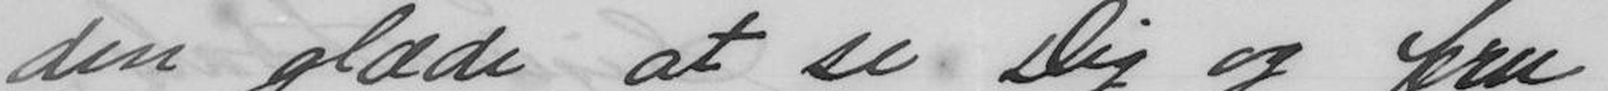den glæde at se Dig og fru
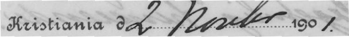Kristiania d. 2 Novbr 1901.
